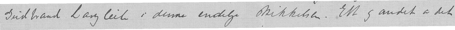Gudbrand Langleite i denne endelige skikkelsen. Ett og andet av det
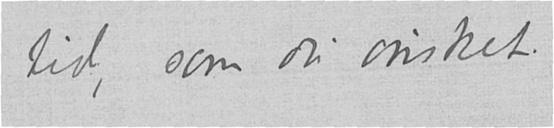tid, som du ønsket.
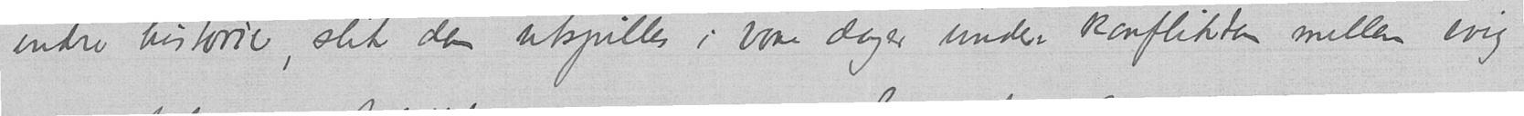indre historie, slik den utspilles i vore dager under konflikten mellem evig
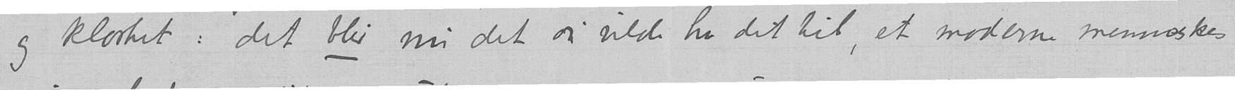og klarhet: det blir nu det du vilde ha det til, et moderne menneskes
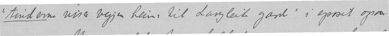"tinderne viser vegjen heim, til Langleite gard" i eposet ogsaa.
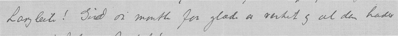Langleite! Gud du maatte faa glæde av verket og al den hæder
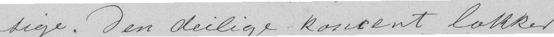sige. Den deilige koncert lokker
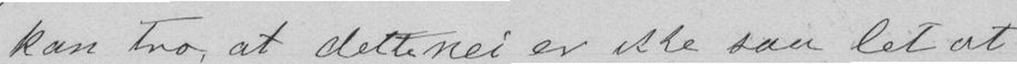kan Tro, at dette nei er ikke saa let at
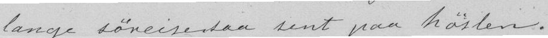lange søreise saa sent paa høsten.
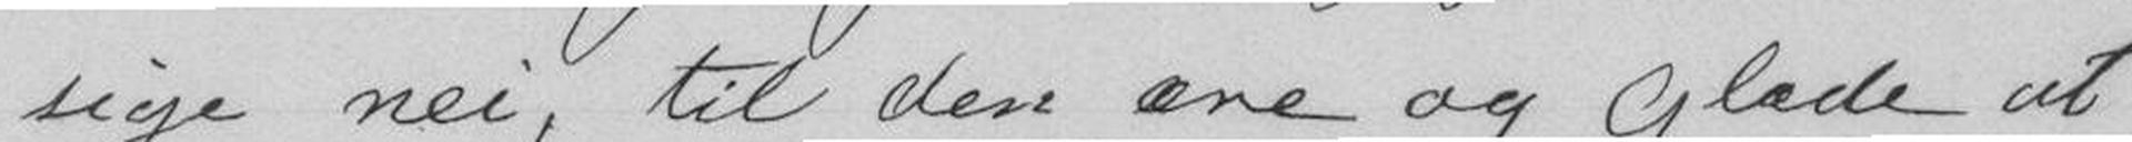sige nei, til den ære og Glæde at
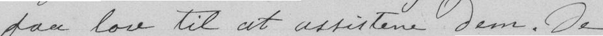faa lov til at assistere Dem. De
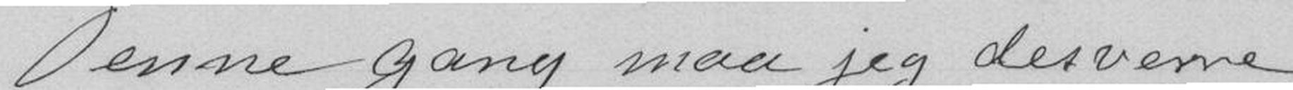Denne gang maa jeg desverre
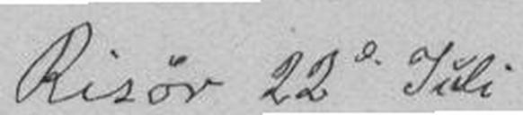Risør 22de Juli
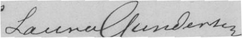Laura Gundersen
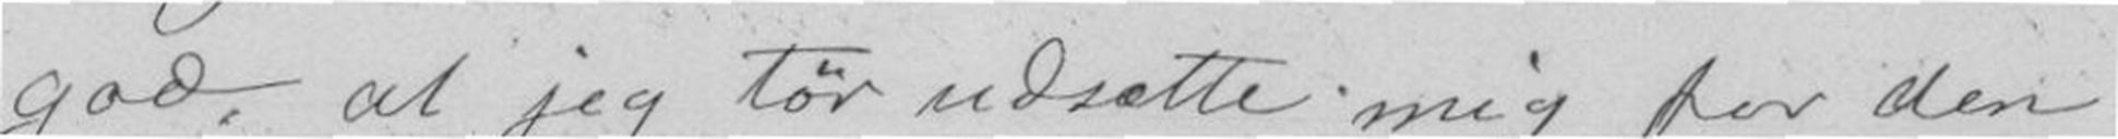god, at jeg Tør udsætte mig for den
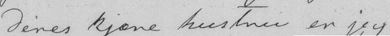Deres kjære hustru er jeg
